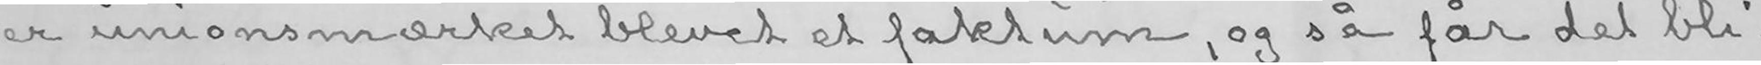er unionsmærket blevet et faktum, og så får det bli-
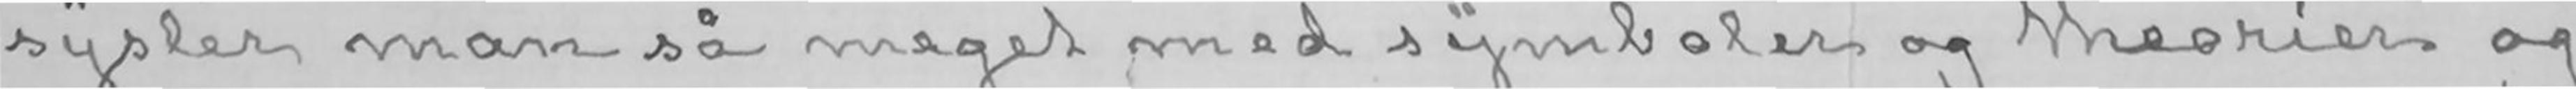sysler man så meget med symboler og theorier og
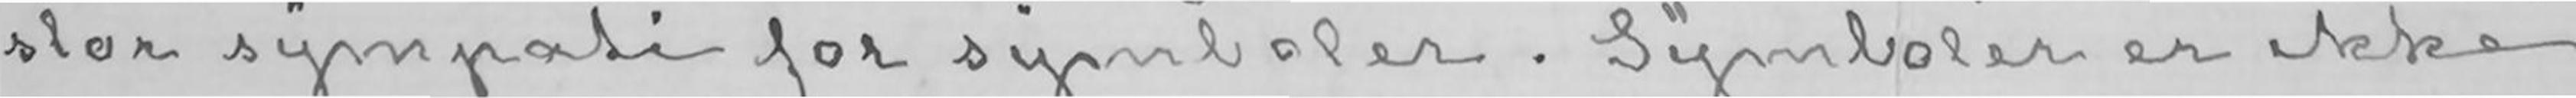stor sympati for symboler. Symboler er ikke
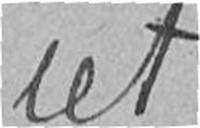let
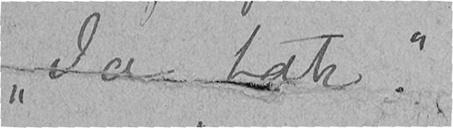"Ja tak."
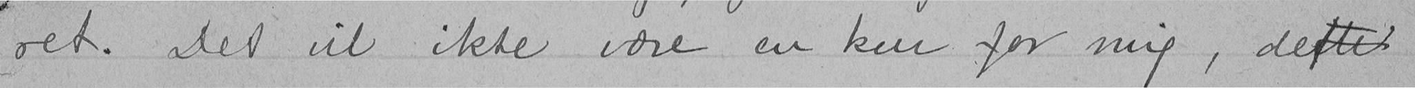ret. Det vil ikke være en kur for mig, dette
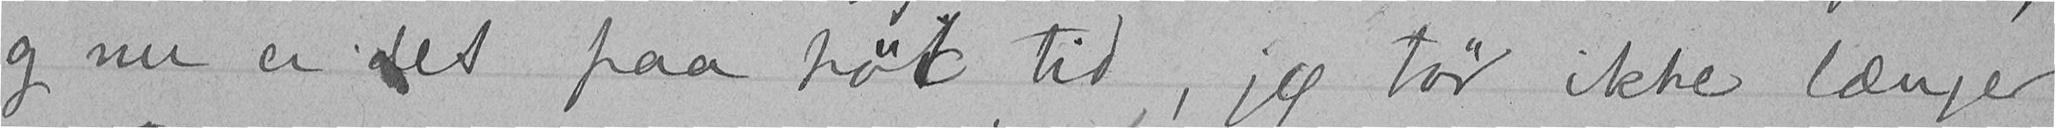og nu er det paa høi tid, jeg tør ikke længer
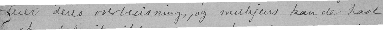terer deres overbevisning, og muligens kan de have
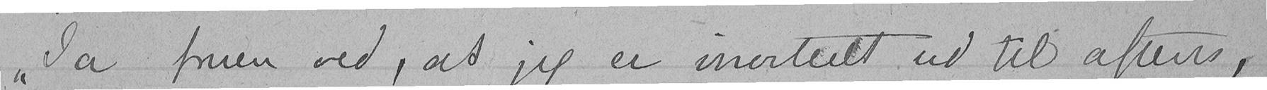"Ja fruen ved, at jeg er inviteret ud til aftens,
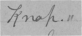Knop."
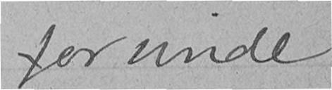forvinde
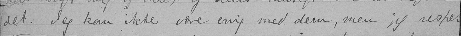det. Jeg kan ikke være enig med dem, men jeg respek-
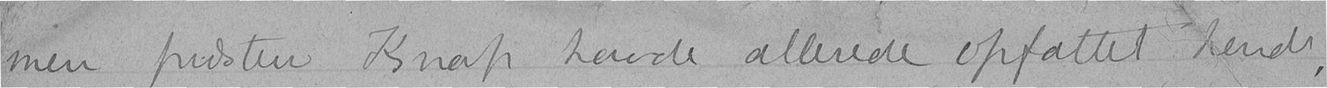men præsten Knop havde allerede opfattet hende,
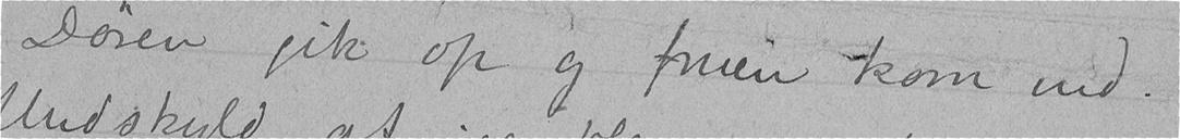Døren gik op og fruen kom ind.
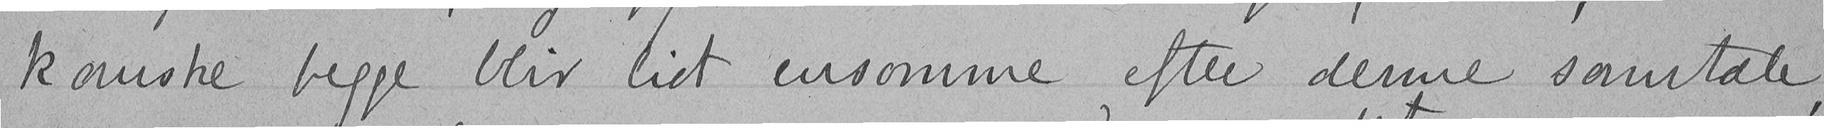kanske begge blir lidt ensomme efter denne samtale,
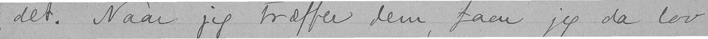det. Naar jeg træffer dem, faar jeg da lov
